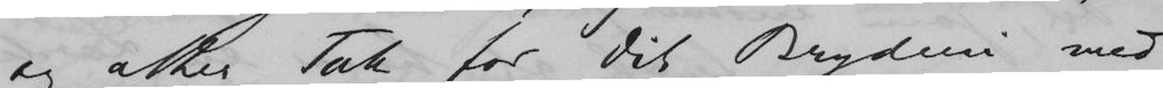og atter Tak for dit Bryderi med
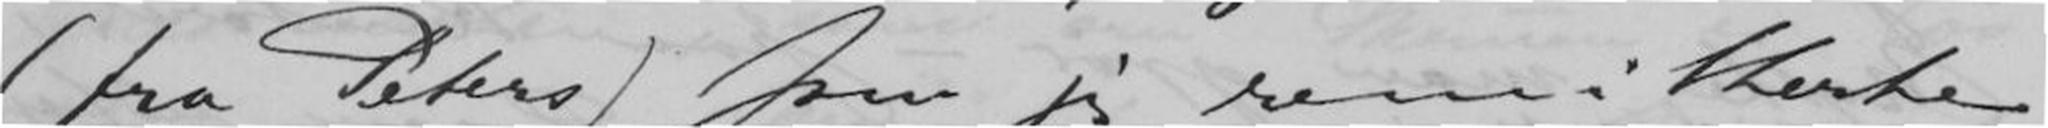(fra Peters) som jeg remitterte
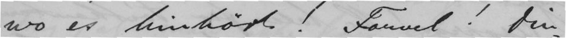wo es hinhört! Farvel! Din
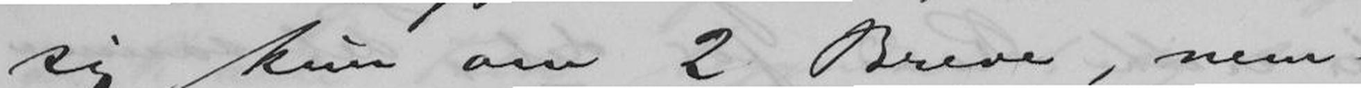sig kun om 2 Breve, nem-
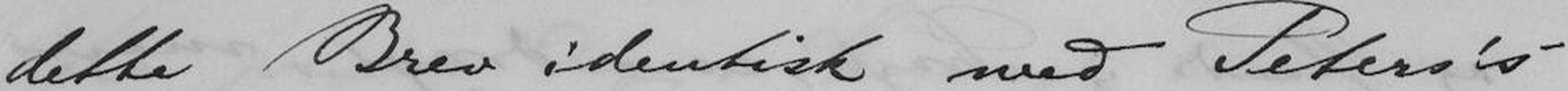dette Brev identisk med Peters's?
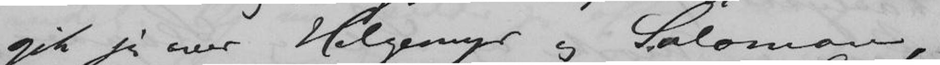gik jeg over Helgemyr og Salomon, -
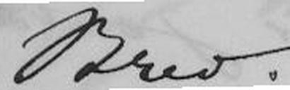Brev. -
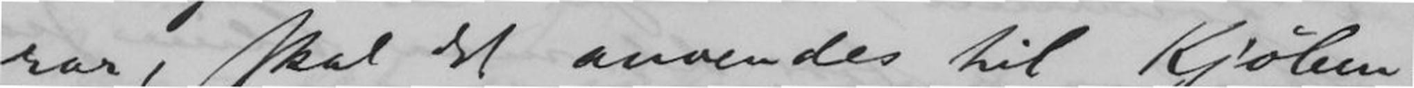rar, skal det anvendes til Kjøben-
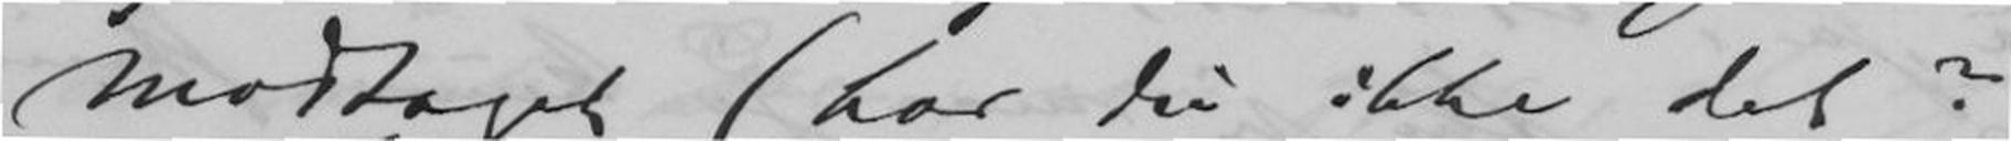modtaget (har du ikke det?)
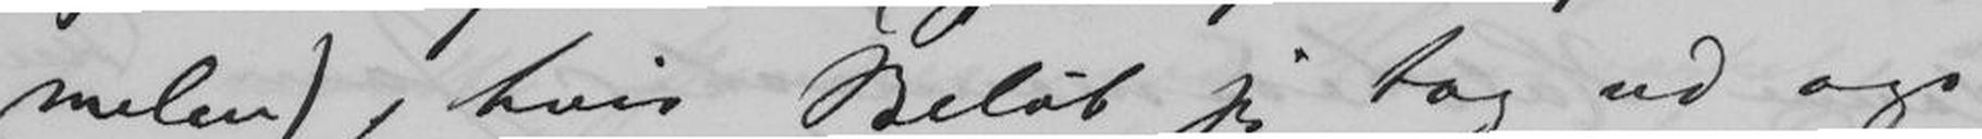melen), hvis Beløb jeg tog ud og
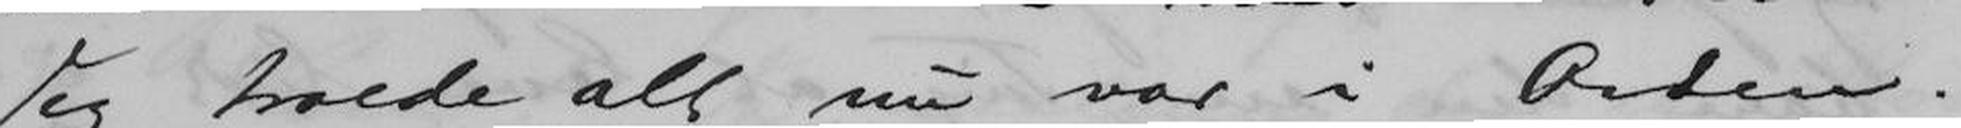Jeg troede alt nu var i Orden.
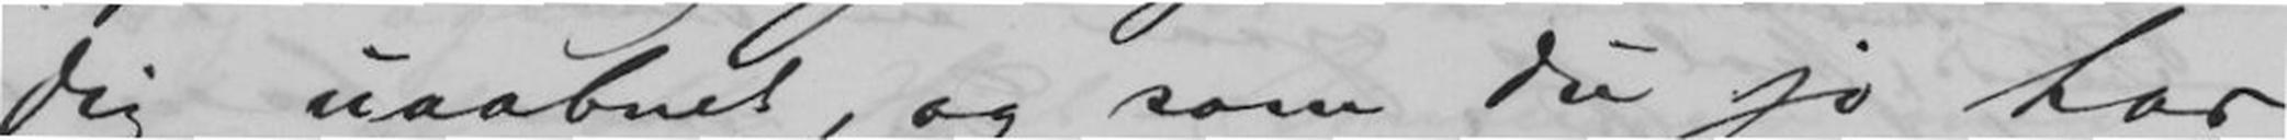dig uaabnet, og som du jo har
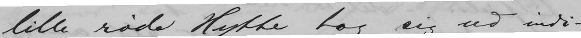lille røde Hytte tog sig ud indi-
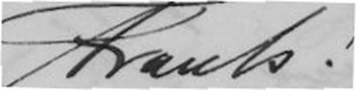Frants!
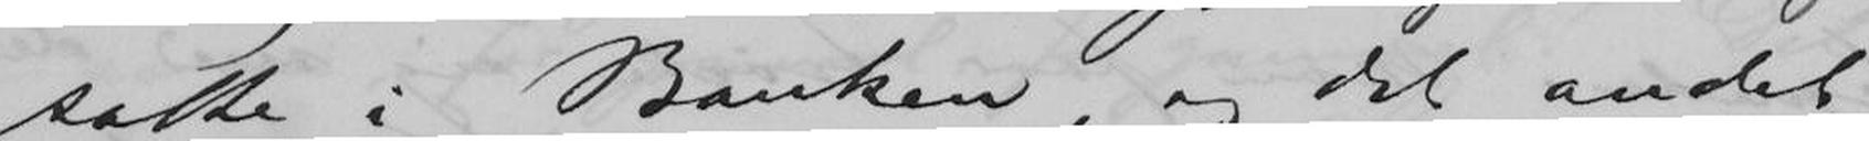satte i Banken, og det andet
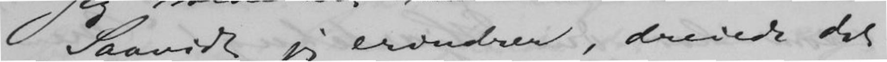Saavidt jeg erindrer, dreiede det
